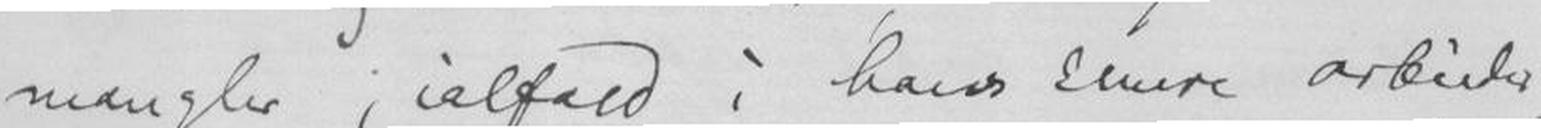mangler, ialfald i hans senere arbeider,
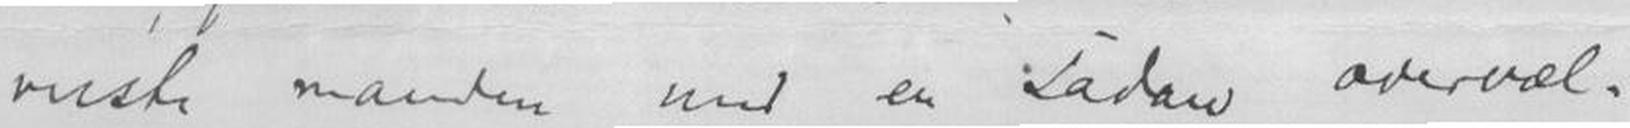ruste manden med en sådan overvæl-
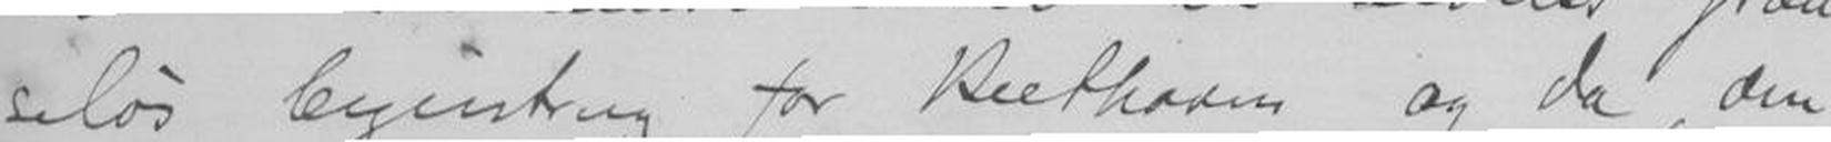seløs begeistring for Bethoven og da, den
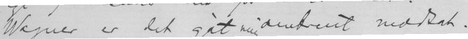Wagner er det gåt mig omtrent modsat.
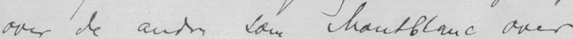over de andre som Montblanc over
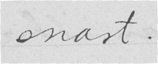snart.
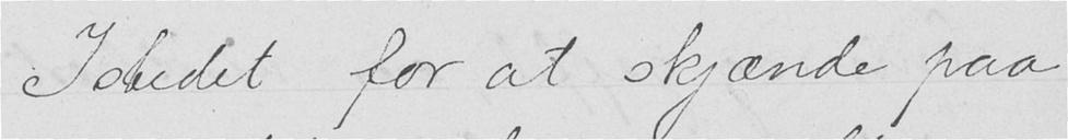Istedet for at skjænde paa
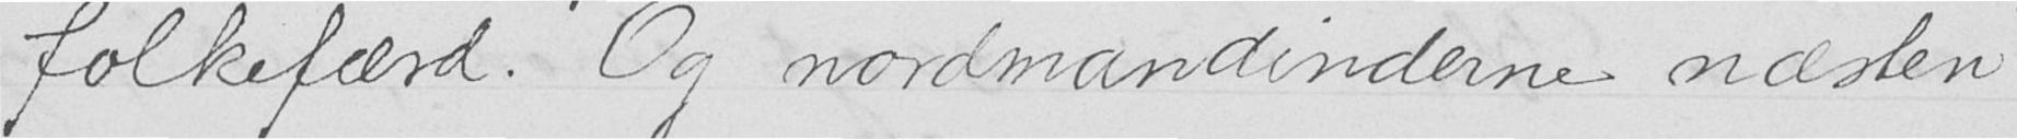folkefærd. Og nordmandinderne næsten
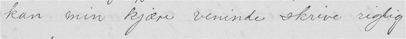kan min kjære veninde skrive rigtig
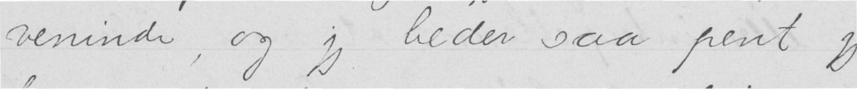veninde, og jeg beder saa pent jeg
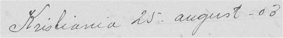Kristiania 25. august -03
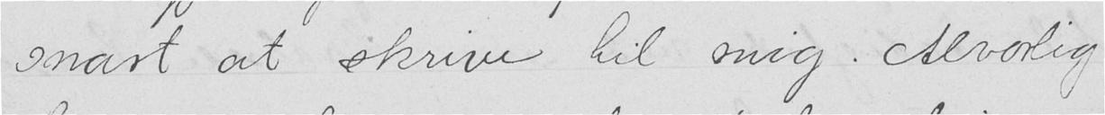snart at skrive til mig. Alvorlig
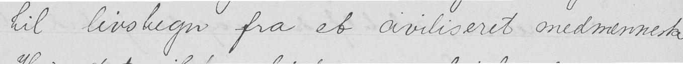til livstegn fra et civiliseret medmenneske.
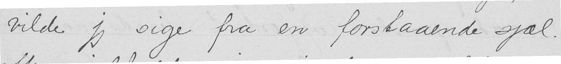vilde jeg sige fra en forstaaende sjæl.
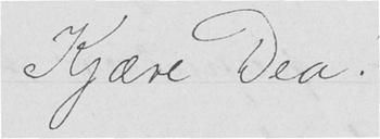Kjære Dea!
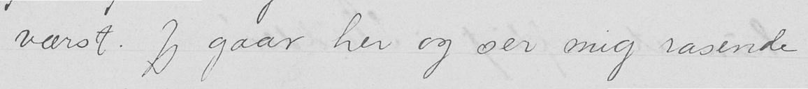værst. Jeg gaar her og ser mig rasende
